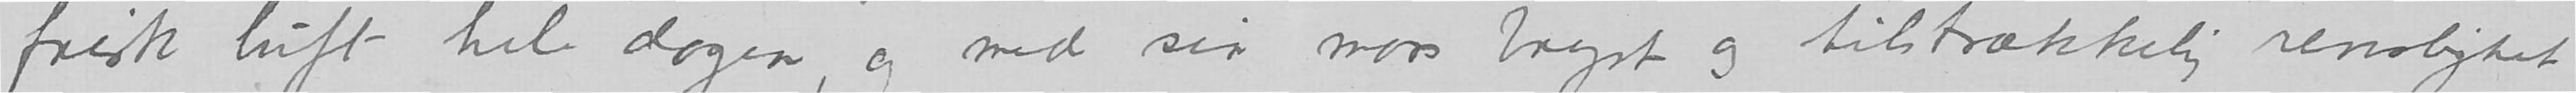frisk luft hele dagen, og med sin mors bryst og tilstrækkelig renslighet
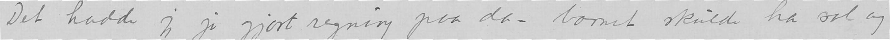Det hadde jeg jo gjort regning paa da – barnet skulde ha sol og
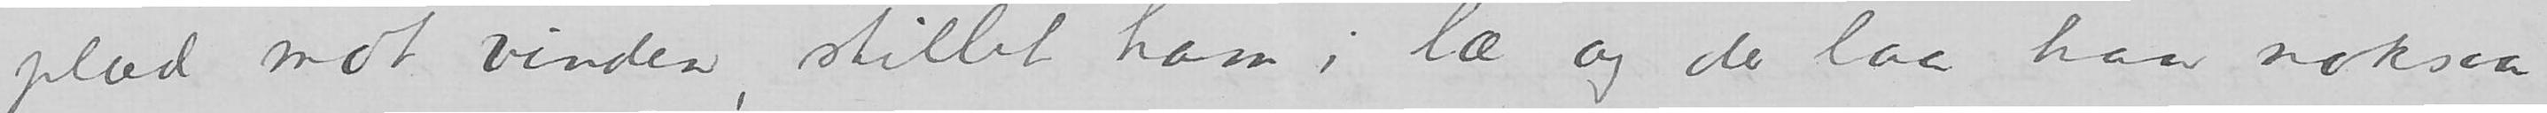plæd mot vinden, stillet ham i læ og der laa han noksaa
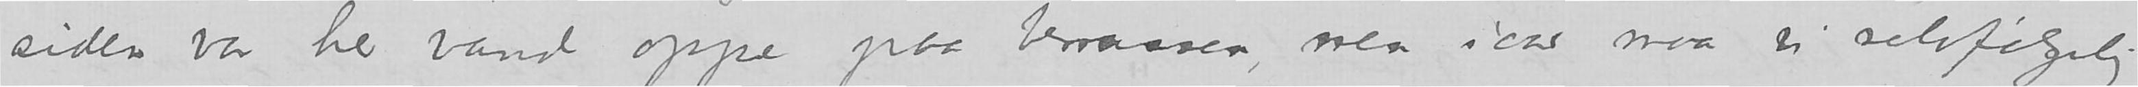siden var her vand oppe paa terrassen, men iaar maa vi selvfølgelig
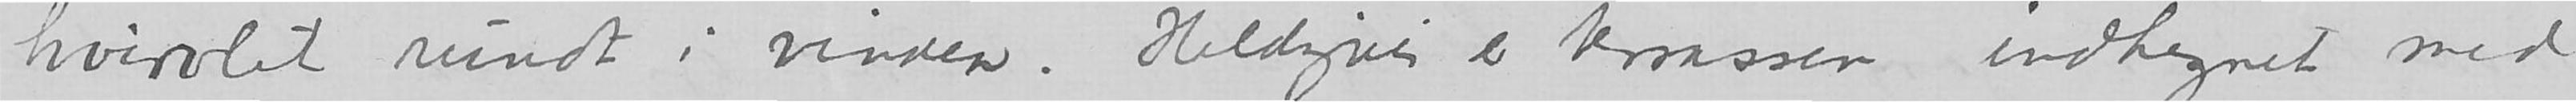hvirvlet rundt i vinden. Heldigvis er terrassen indhegnet med
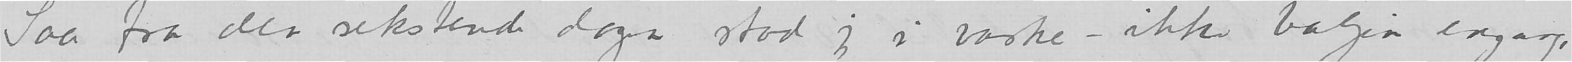Saa fra den sekstende dagen stod jeg i vaske – ikke baljen engang,
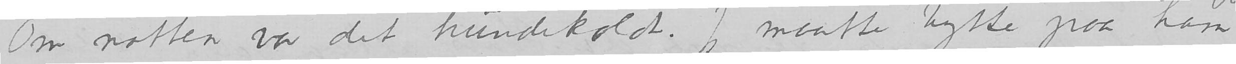Om natten var det hundekoldt. Jeg maatte bytte paa ham
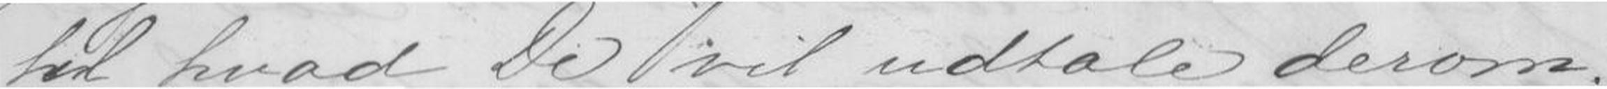til hvad De vil udtale derom;
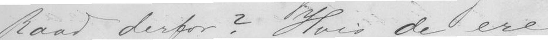Raad derfor? Hvis de ere
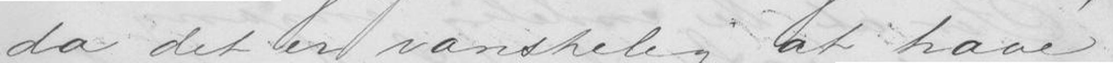da det er vanskelig at haave
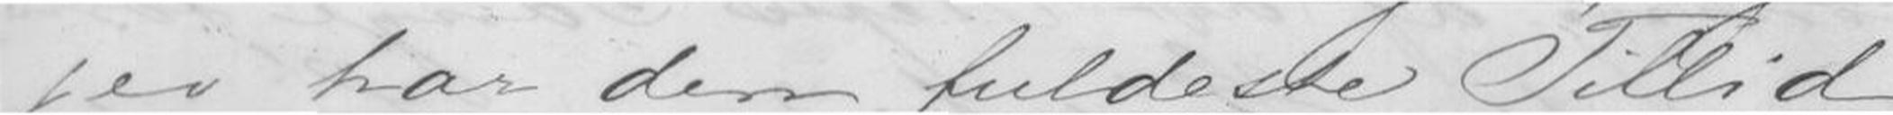jeg har den fuldeste Tillid
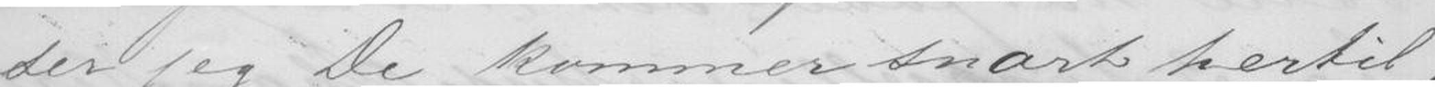ser jeg De kommer snart hertil,
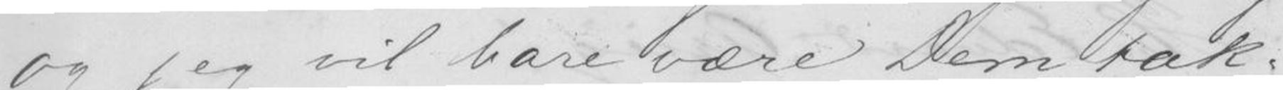og jeg vil bare være Dem tak-
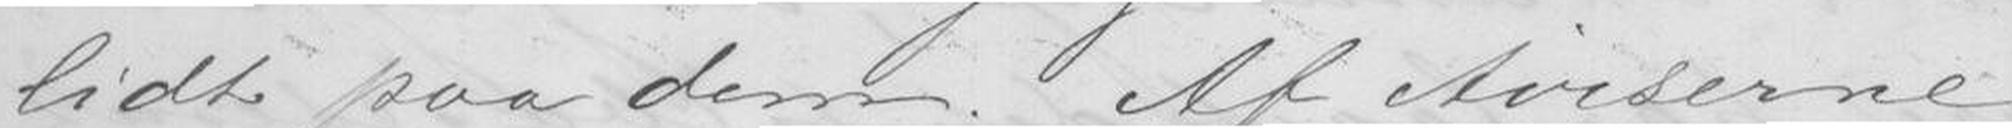lidt paa dem. Af Aviserne
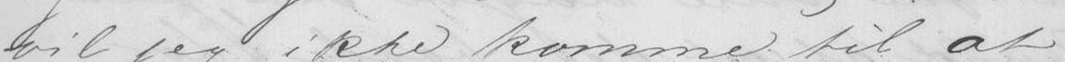vil jeg ikke komme til at
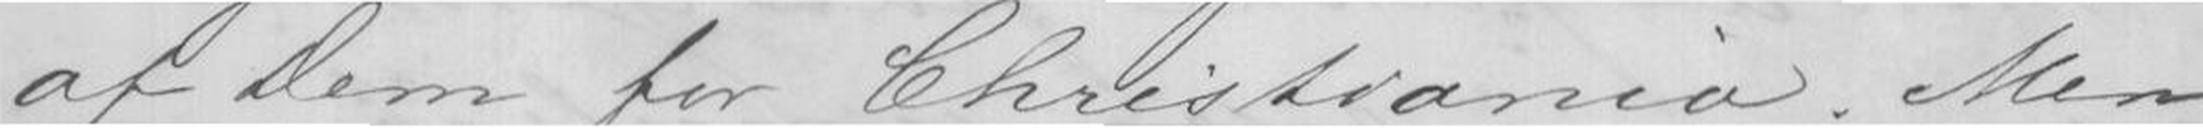af Dem for Christiania. Men
